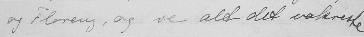og Florens, og se alt det vakreste
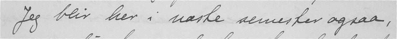Jeg blir her i næste semester ogsaa,
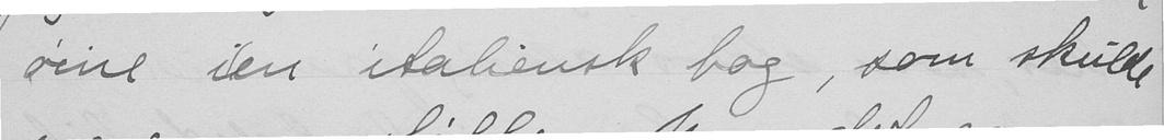øine ien italiensk bog, som skulde
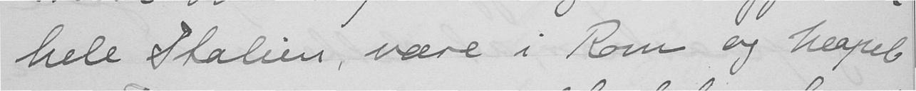hele Italien, være i Rom og Neapel
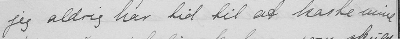jeg aldrig har tid til at kaste mine
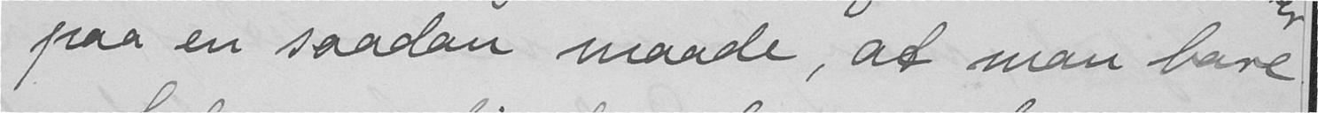paa en saadan maade, at man bare
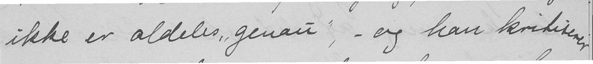ikke er aldeles "genau"- og han kritiserer
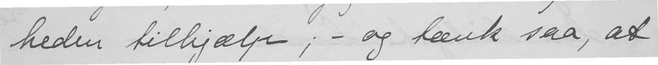heden tilhjælp,- og tænk saa, at
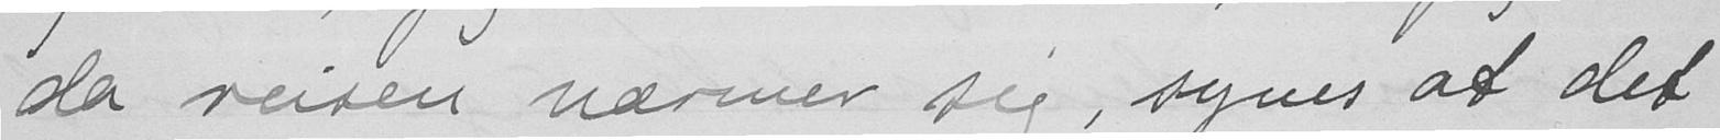da reisen nærmer sig, synes at det
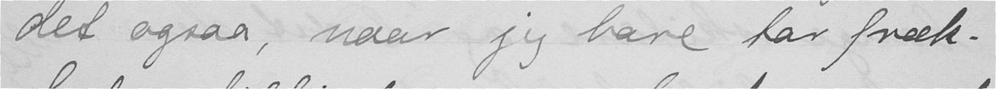det ogsaa, naar jeg bare tar fræk-
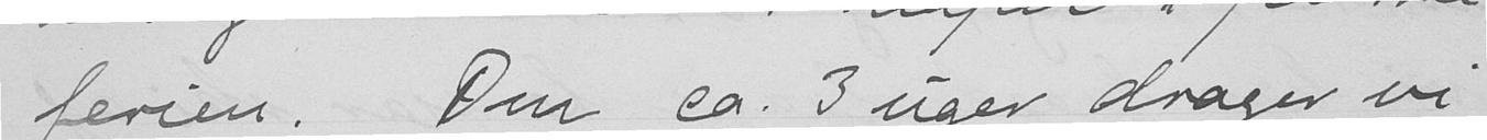ferien. Om ca. 3 uger drager vi
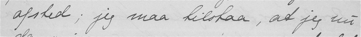afsted; jeg maa tilstaa, at jeg nu
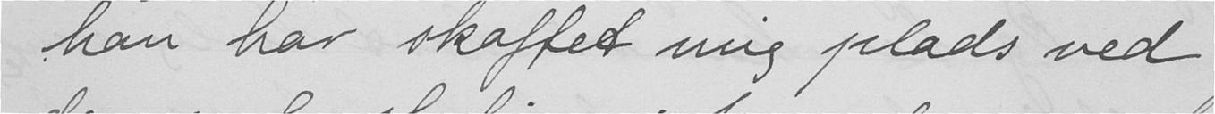han har skaffet mig plads ved
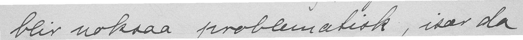blir noksaa problematisk, især da
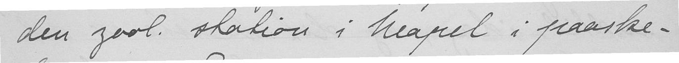zool. station i Neapel i paaske-
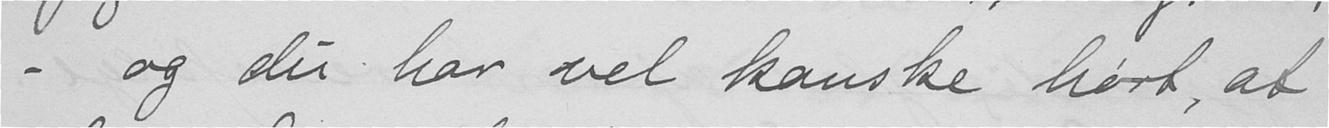- og du har vel kanske hørt at
